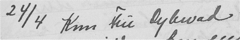24/4 Kom Fru Dybwad
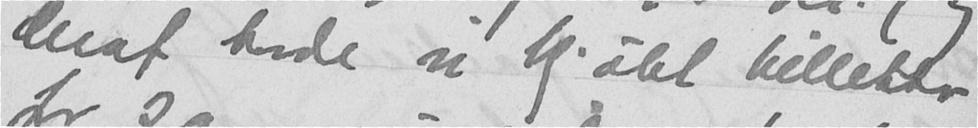deraf havde vi kjøbt billetter
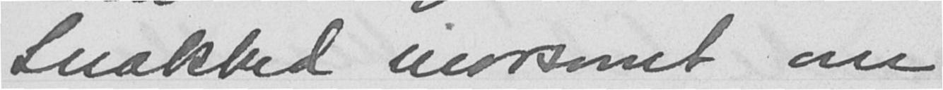Snakked morsomt om
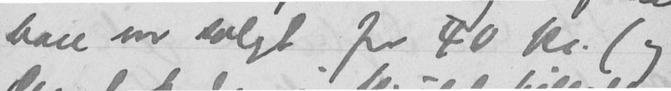bare var solgt for 40 kr. (og
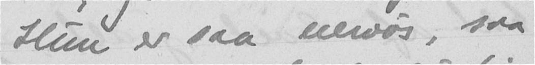Hun er saa nervøs, saa
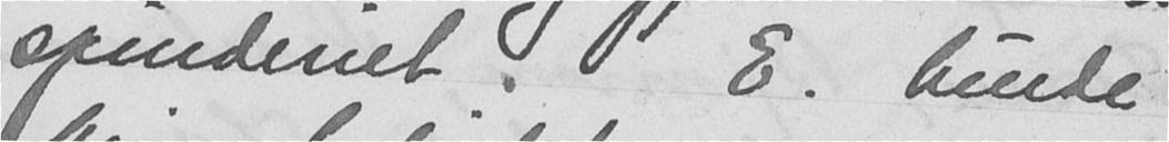spinderiet. E. burde
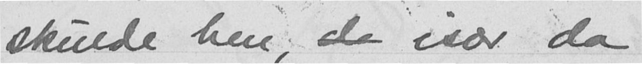skulde hen, da især da
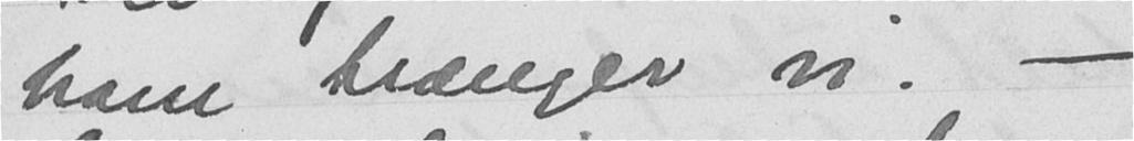ham trænger vi. -
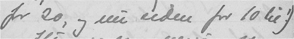for 20, og nu siden for 10 kr!)
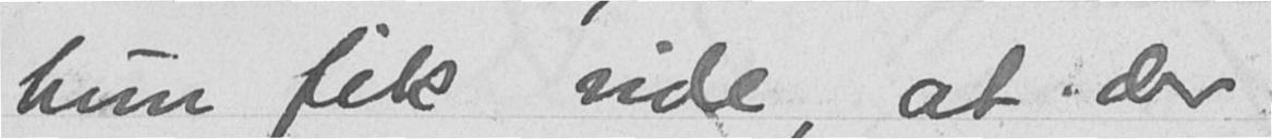hun fik vide, at der
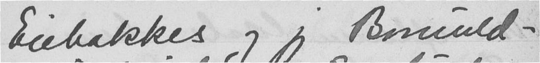Eiebakkes og jeg Bomuld-
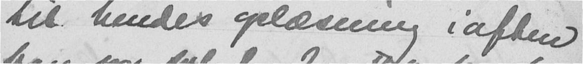til hendes oplæsning i aften
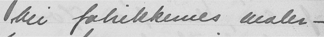bli fabrikkernes maler -
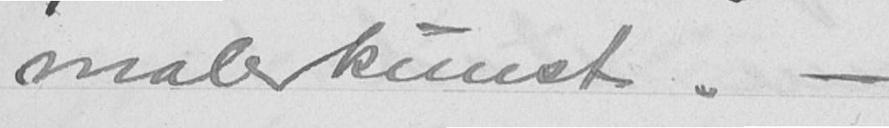malerkunst. -
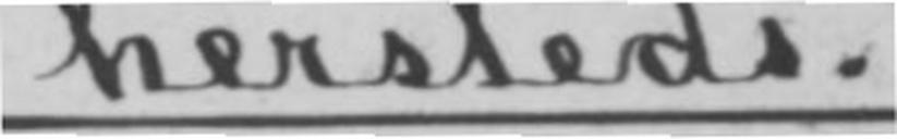hersteds.
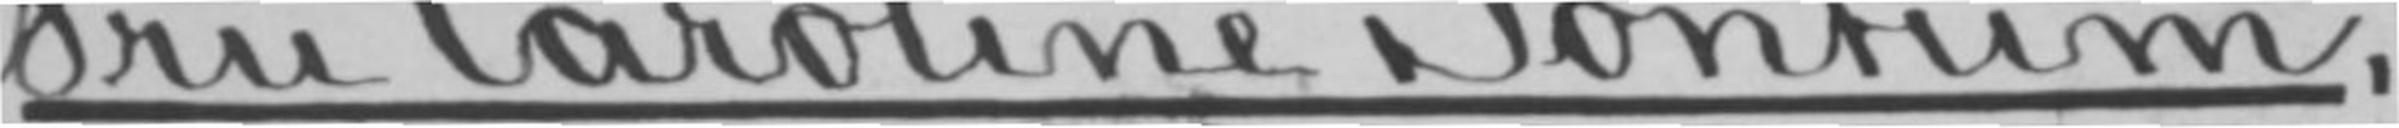Fru Caroline Sontum,
